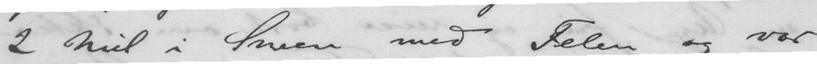2 Mil i Sneen med Felen og var
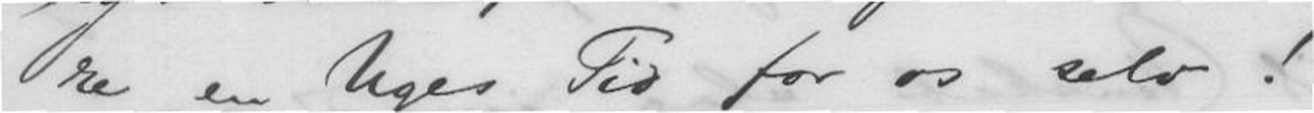re en Uges Tid for os selv!
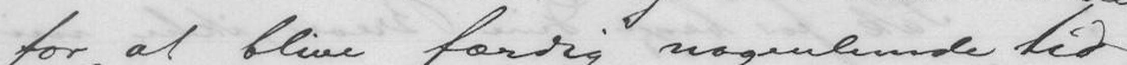for at blive færdig nogenlunde tid-
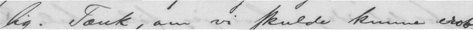lig. Tænk, om vi skulde kunne erob-
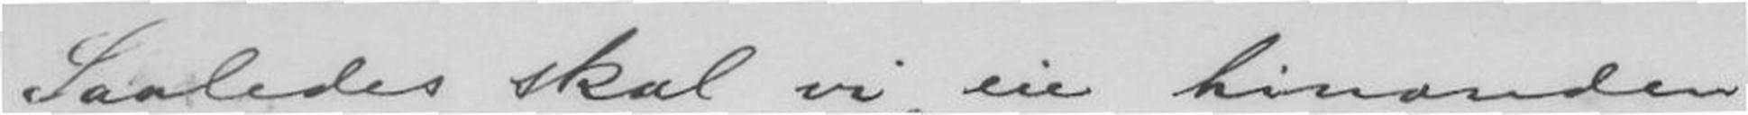Saaledes skal vi eie hinanden
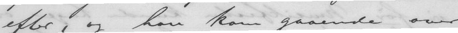efter, og han kom gaaende over
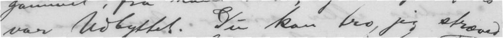var Udbyttet. Du kan tro, jeg strævet
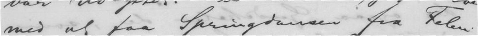med at faa Springdansen fra Felen
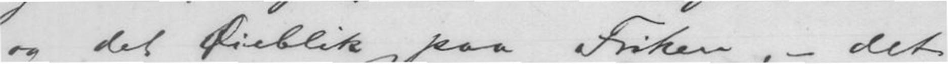og det Øieblik paa Friken, - det
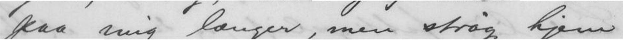paa mig længer, men strøg hjem
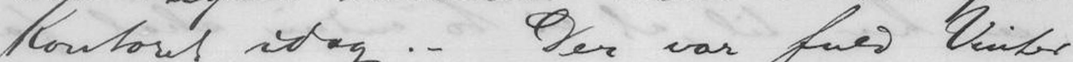Kontoret idag. - Der var fuld Vinter
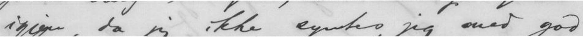igjen, da jeg ikke syntes, jeg med god
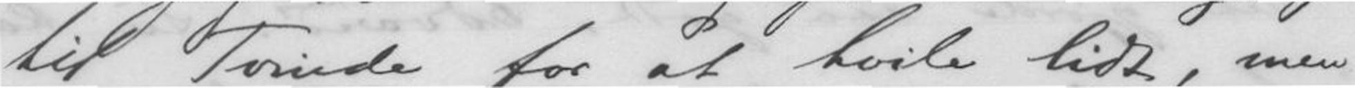til Tvinde for at hvile lidt, men
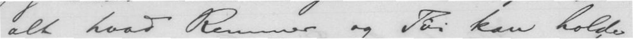alt hvad Remmer og Tøi kan holde
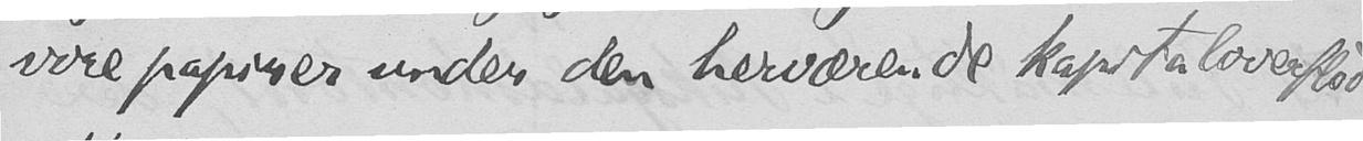vore papirer under den herværende kapitaloverflod
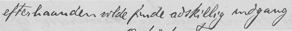efterhaanden vilde finde adskillig indgang.
